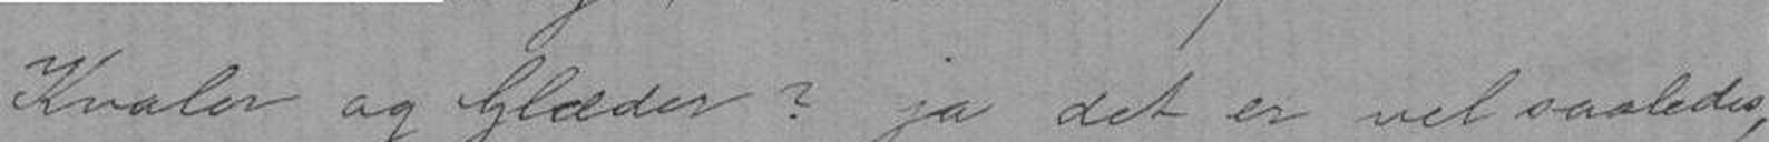Kvaler og Glæder? ja det er vel saaledes,
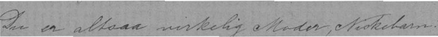Du er altsaa virkelig Moder, Niskebarn!
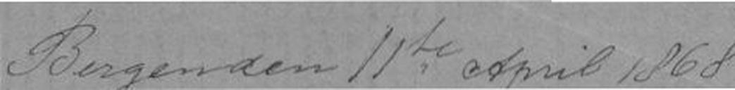Bergen den 11te April 1868.
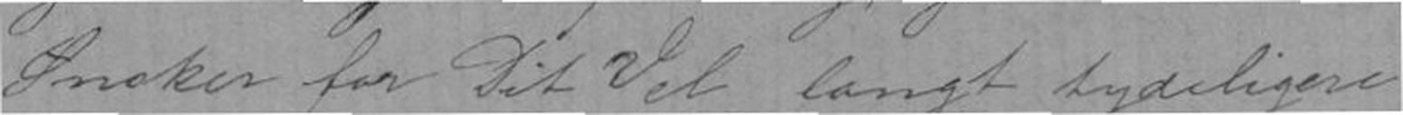Ønsker for Dit Vel langt tydeligere
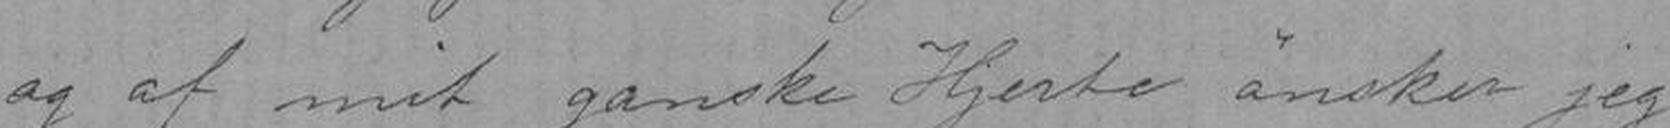og af mit ganske Hjerte ønsker jeg
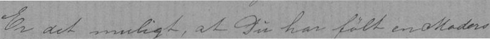Er det muligt, at Du har følt en Moders
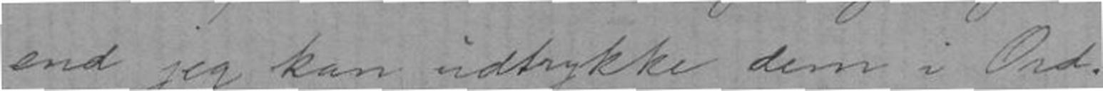end jeg kan indtrykke dem i Ord.
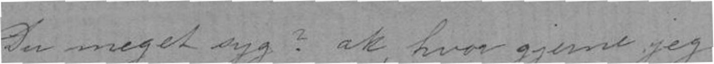Du meget syk? ak, hvor gjerne jeg
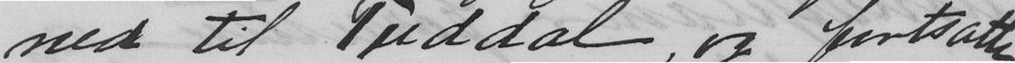ned til Tuddal, og fortsatte
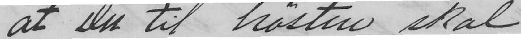at Du til høsten skal
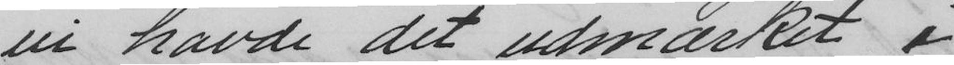vi havde det udmærket i
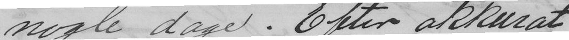nogle dage. Efter akkurat
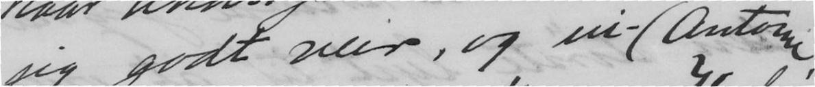jeg godt veir, og vi - (Antonie,
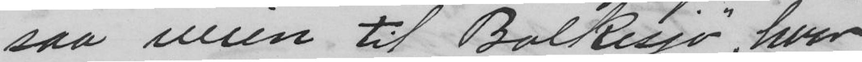saa veien til Bolkesjø, hvor
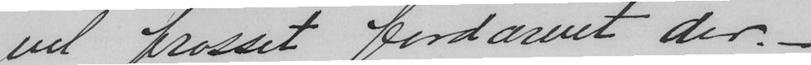vel frosset fordærvet der. -
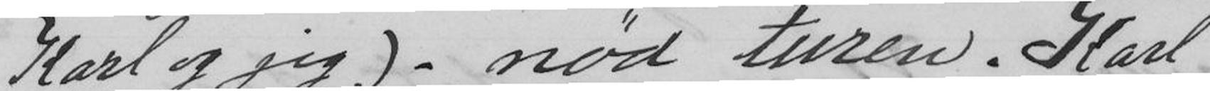Karl og jeg) - nød turen. Karl
